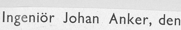Ingeniör Johan Anker, den
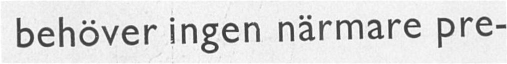behöver ingen närmare pre-
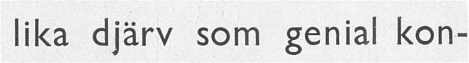lika djärv som genial kon-
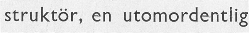struktör, en utomordentlig
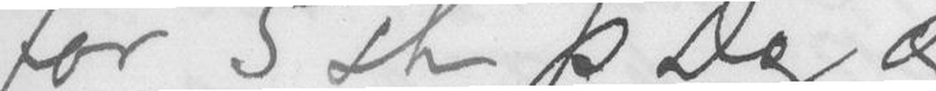for 5 sh p Dag og
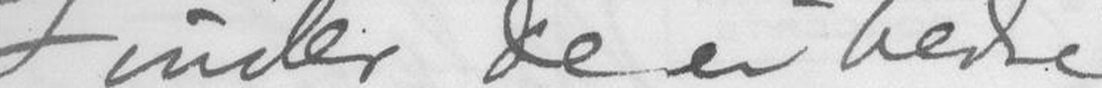Finder de ei bedre,
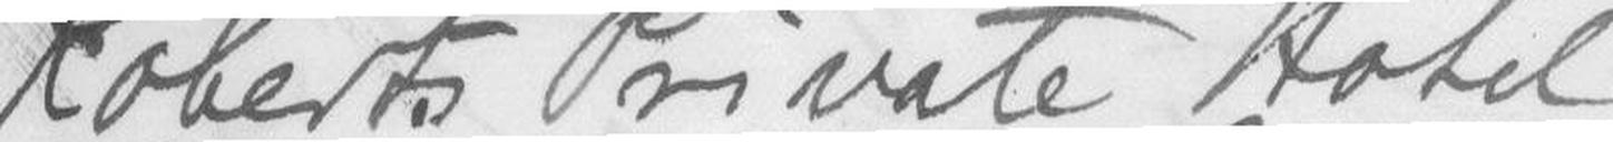Roberts Private Hotel
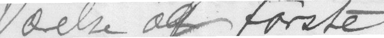Værelse og første
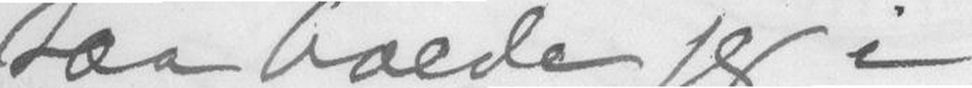saa boede jeg i
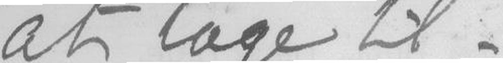at tage til.
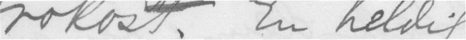Frokost. En heldig
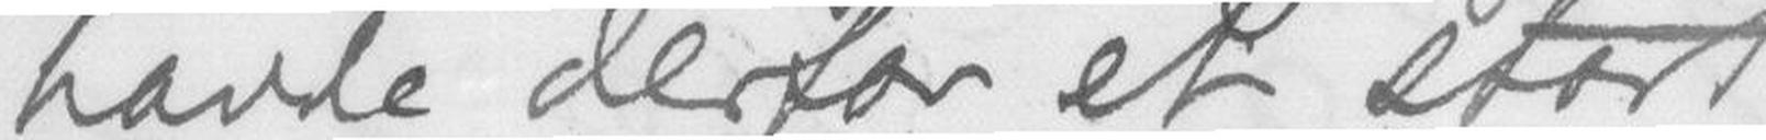havde derfor et stort
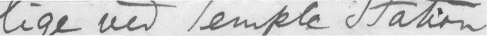lige ved Temple Staion
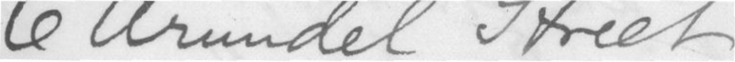6 Arundel Street
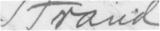Strand
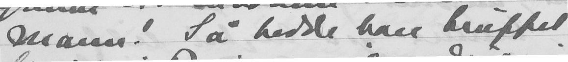mann! Så hadde han truffet
